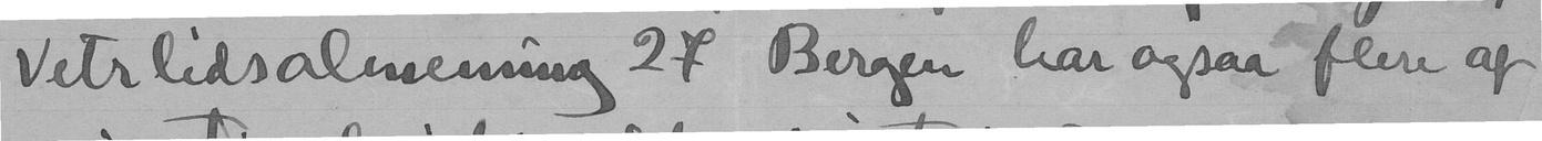Vetrlidsalmenning 27 Bergen har ogsaa flere af
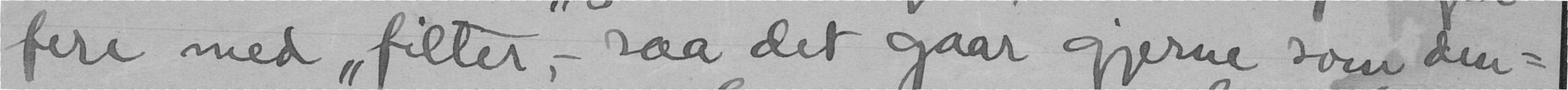fere med "filter", - saa det gaar gjerne som den-
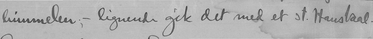himmelen; - lignende gik det med et st. Hansbaal.
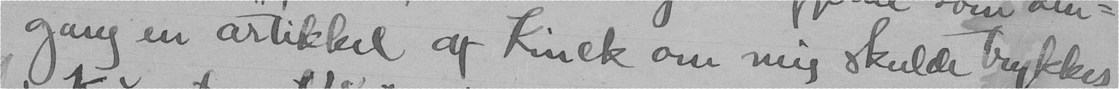gang en artikkel af Kinck om mig skulde trykkes
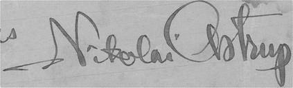Nikolai Astrup
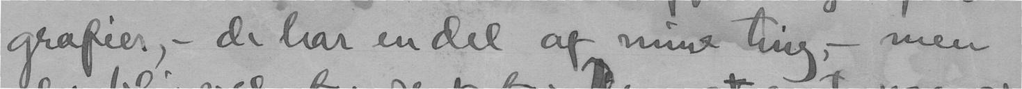grafier, - de har en del af mine ting, - men
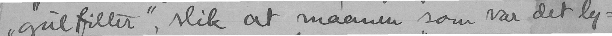"gulfilter" slik at maanen som var det ly-
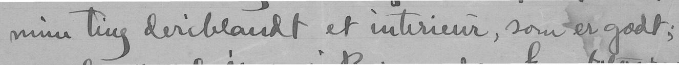mine ting deriblandt et interieur, som er godt;
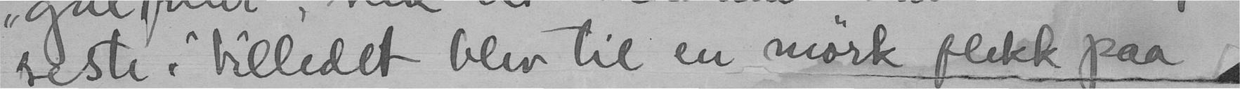seste i billedet blev til en mørk flekk paa
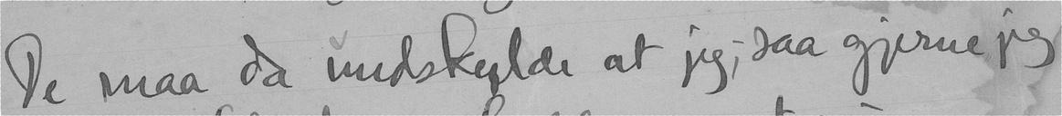De maa da undskylde at jeg, - saa gjerne jeg
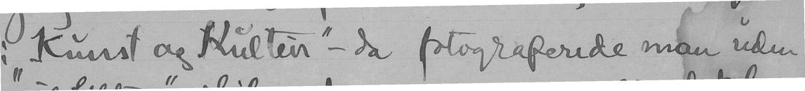i "Kunst og Kultur" - da fotograferede man uden
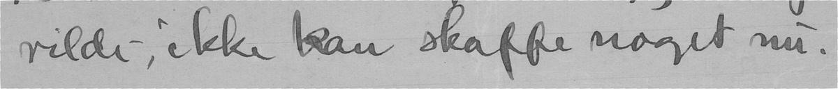vilde -, ikke kan skaffe noget nu.
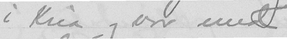i Kria og var med
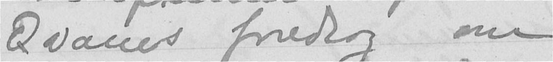Qvanes foredrag om
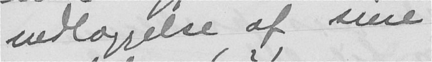nedlæggelse af sine
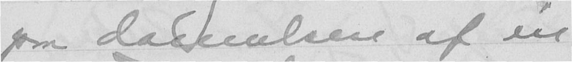paa dannelsen af én
